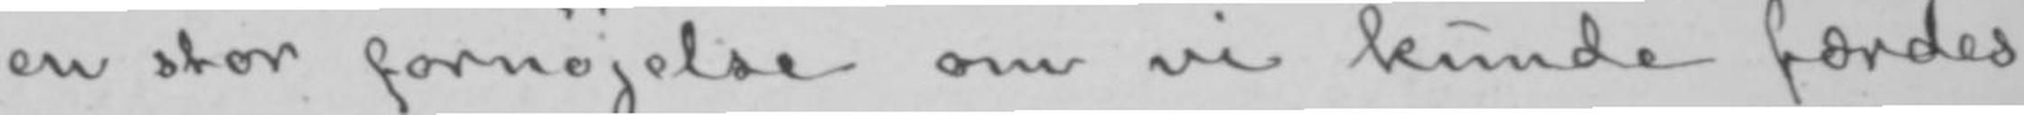en stor fornøjelse om vi kunde færdes
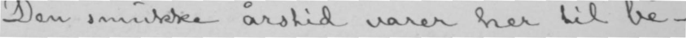Den smukke årstid varer her til be-
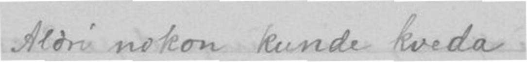Aldrig nokon kunde kveda
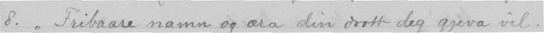8. Fribaare namn og æra din drott deg gjeva vil.
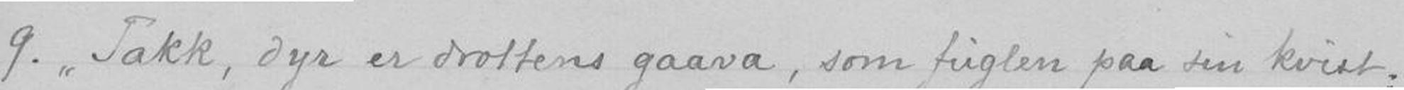9. Takk, dyr er drottens gaava, som fuglen paa sin kvist;
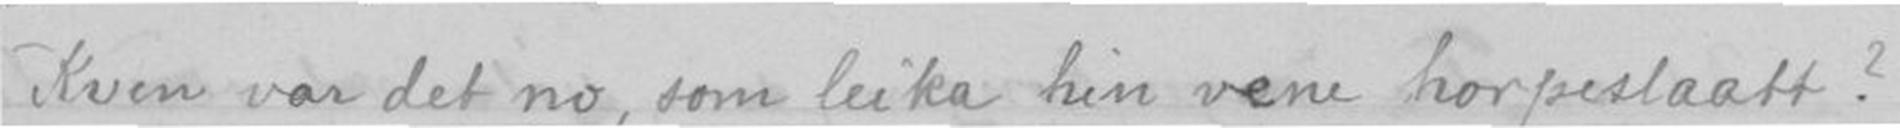Kven var det no, som leika hin væne harpeslaatt?
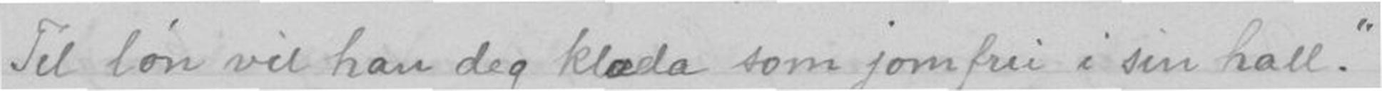Til løn vil han deg klæda som jomfra i sin hall.
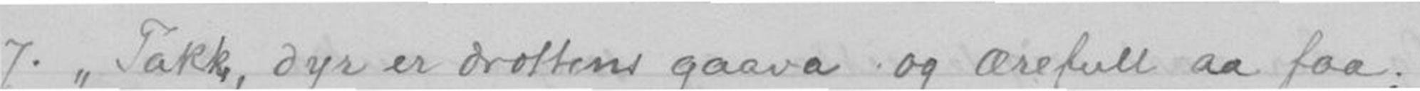7. Takk, dyr er drottens gaava og ærefull aa faa;
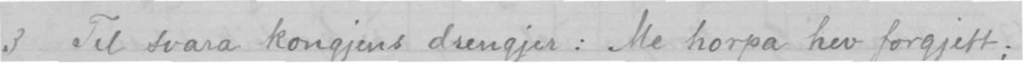3. Til svara kongjens drengjer: Me horpa hev forgjett;
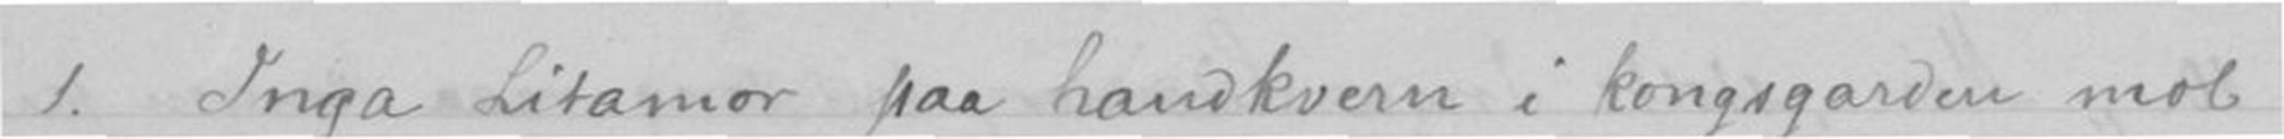1. Inga Litamor paa handkvern i kongsgarden mol
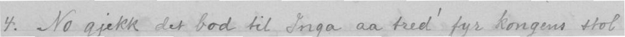4. No gjekk det bod til Inga aa tred' fyr kongens stol
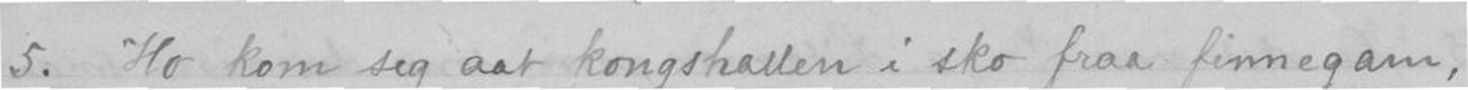5. Ho kom seg aat kongshallen i sko fraa finnegarn,
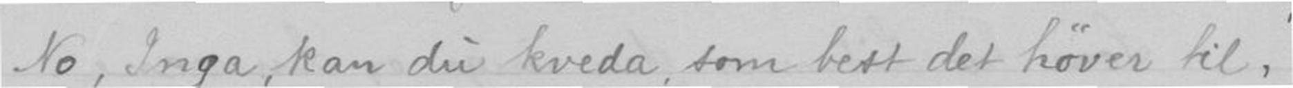No, Inga, kan du kveda som best det høver til.
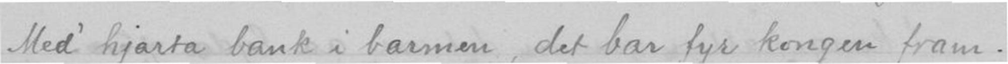Med hjarta bank i barmen, det bar fyr kongen fram.
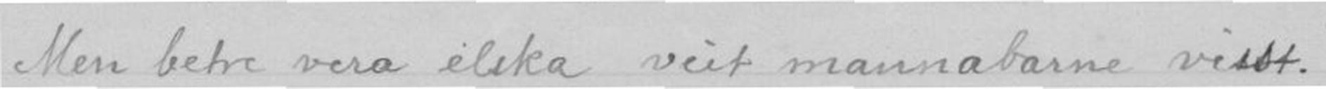Men betre vera elska veit mannabarne visst.
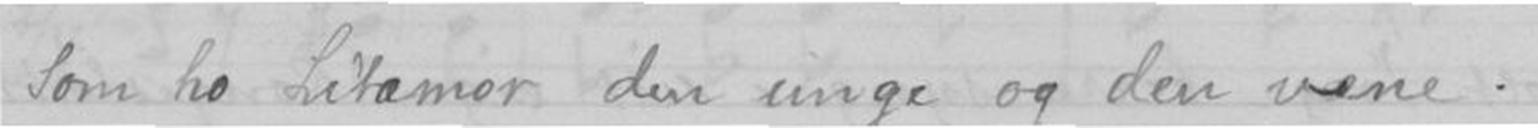Som ho Litamor den unge og den væne.
